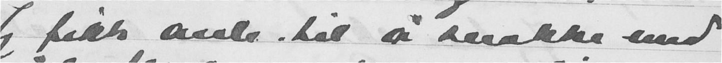Jeg fikk anl. til a snakke med
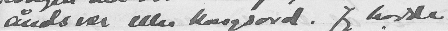åndsvær eller kongsord. Jeg hadde
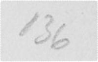136
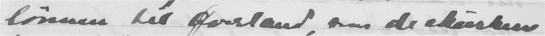lønnen til Øvarland, som de skurkene
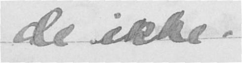de ikke.
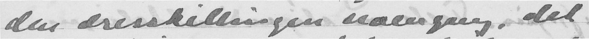den æresskillingen noengang, det
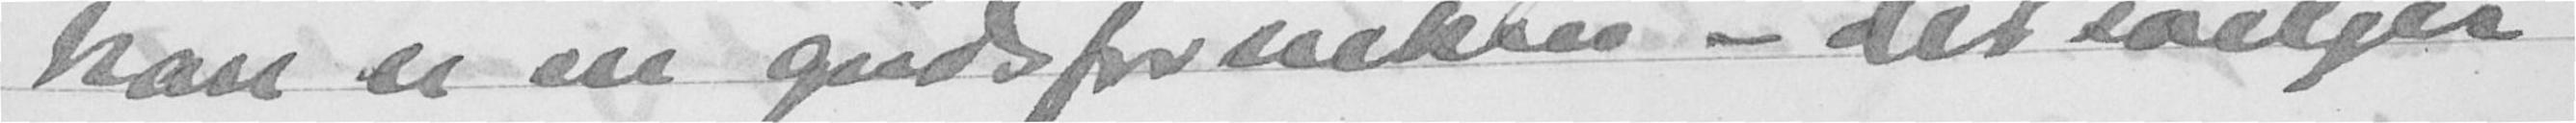han er en gudsfornekter - det velger
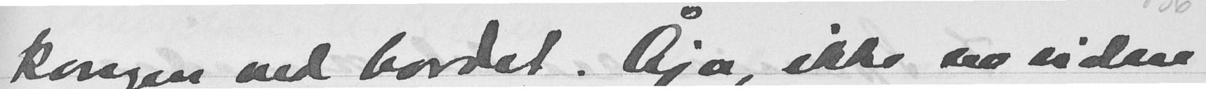kongen ved bordet. Åja, ikke no videre
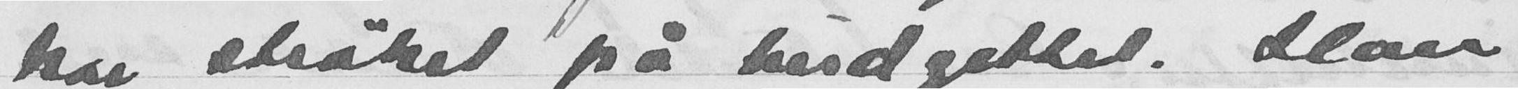har strøket på budsjettet. Han
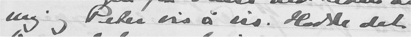mig og Peter vis a vis. Hadde det
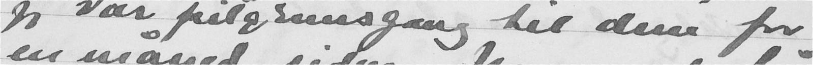jeg var pilgrimsgang til dem for
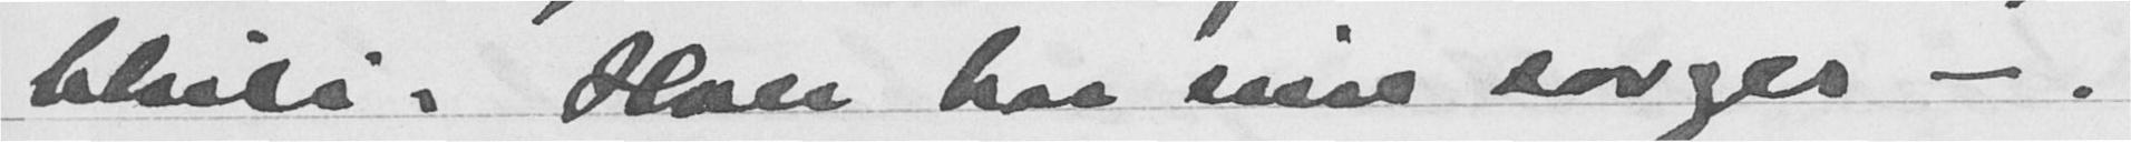Bhili. Han har sine sorger -.
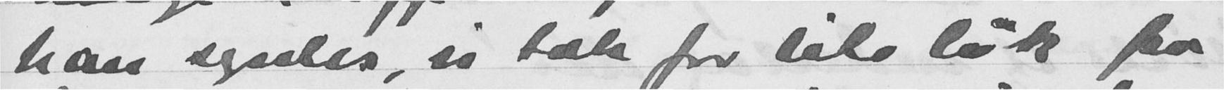han syntes, vi tok for lite løk fra
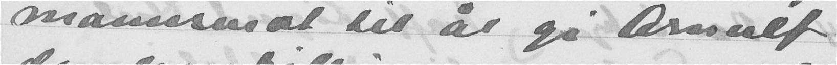mannsmot til å gi Arnulf
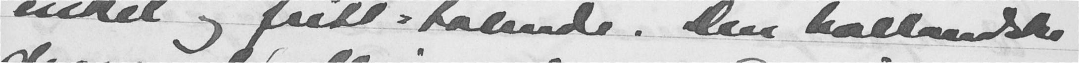enkel og fritt-talende. Den hollandske
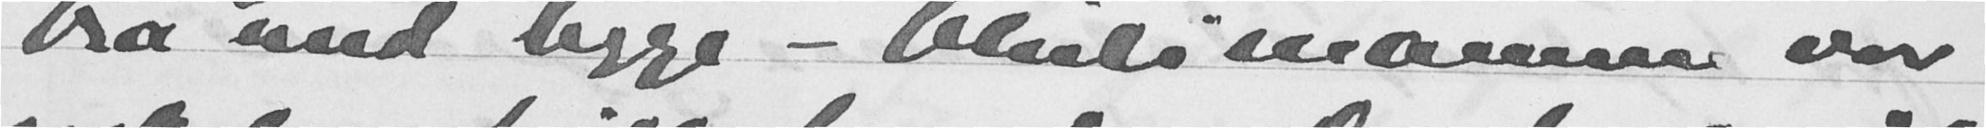bra med begge - Bhilimannen var
